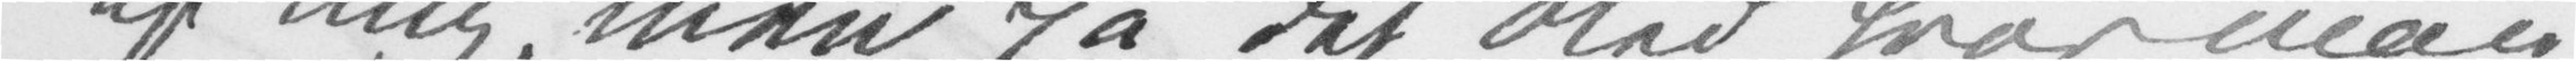af mig, men på det Sted hvor man
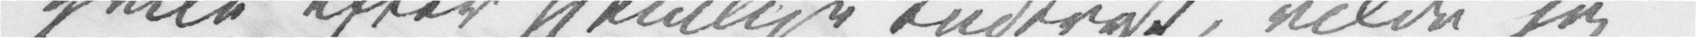Hvile efter hjemlige Indtryk, vilde jeg
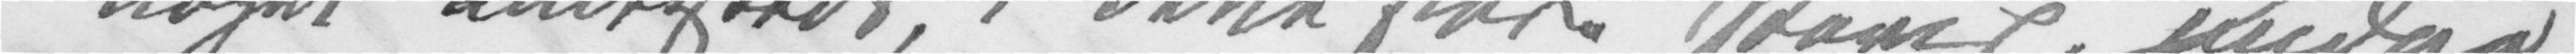noget andetsteds, i dette store Paris, undgå
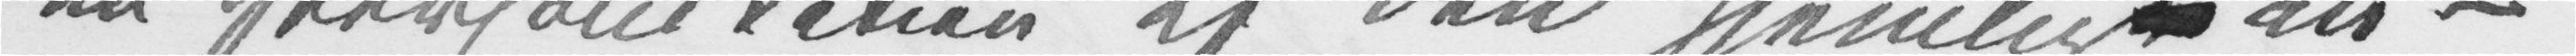en Personi[fi]kation af den hjemlig t au¬
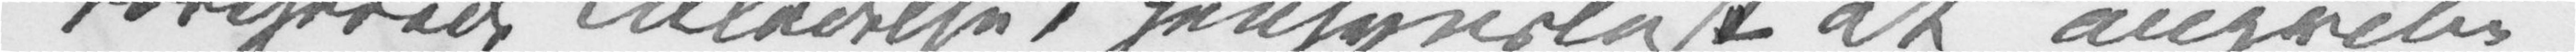toriserede Tilladelse, hensynsløst at angribe
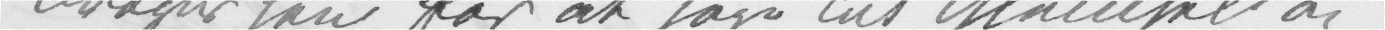drager hen for at søge lidt Glemsel og
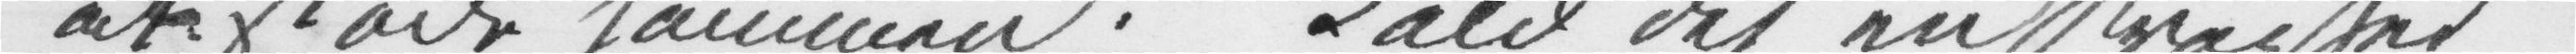at støde sammen. Kald det en Svaghed
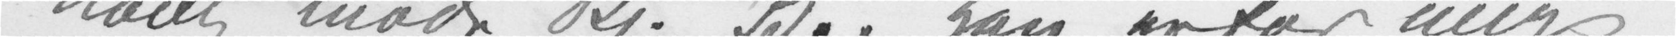nødig møde Bj. Bj. Han er for mig
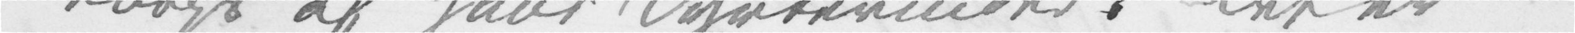Korps af hans Dyrkerinder. Det er
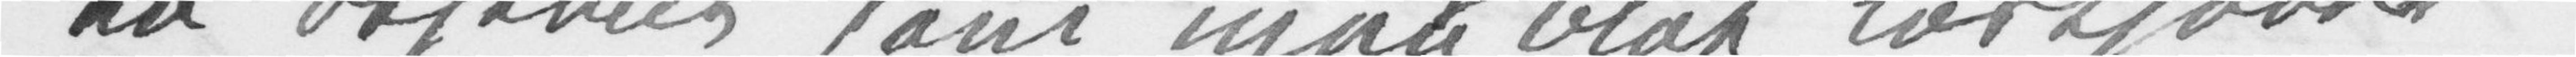en Skjebne som man blot løskjøber
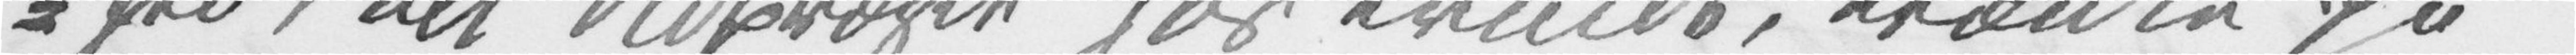hed, alt Udpræget hos Kvinde, krænke på
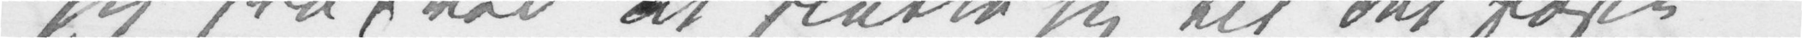sig fra, ved at slutte sig til det faste
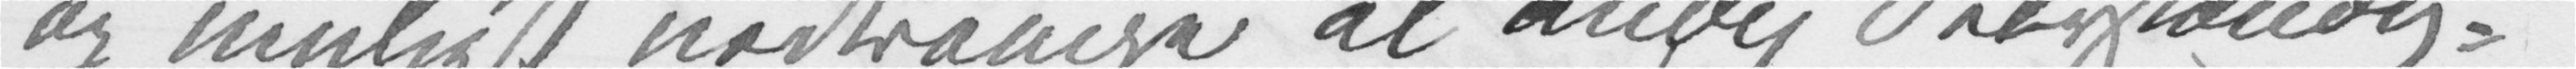og muligst nedtrampe al åndig Selvstændig¬
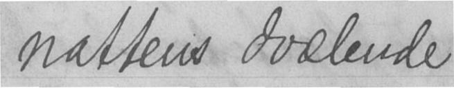nattens dvælende
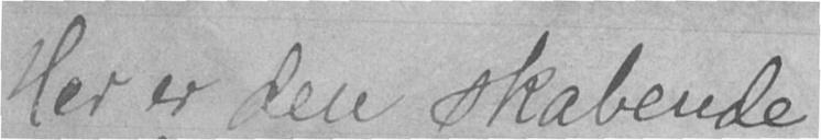Her er den skabende
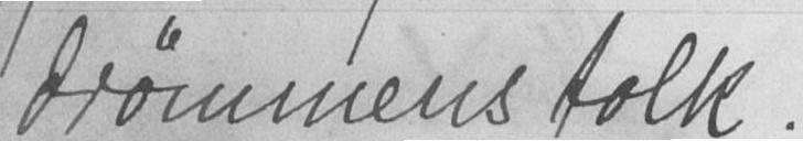drømmens folk.
